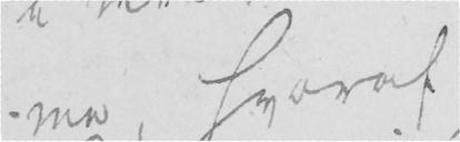en hvoraf
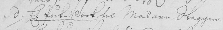"- d " Et Puk-Værk til Masovn-Slaggen.
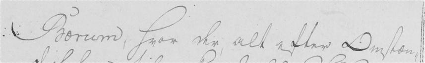Bærum, hvor der alt efter Omstæn-
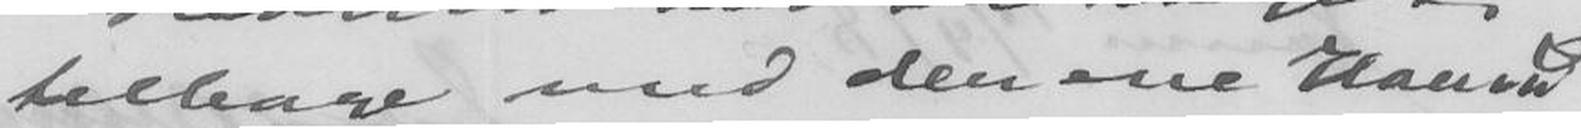tilbage med den ene Haand
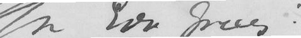Hr Edv Grieg!
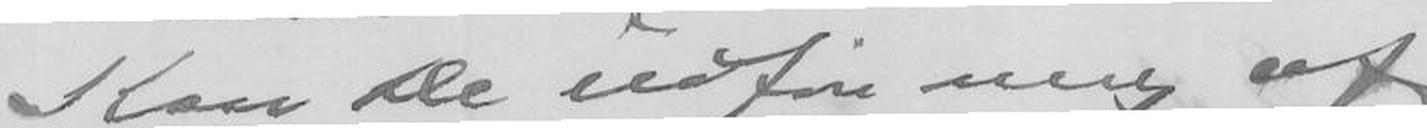Kan De udføre mig af
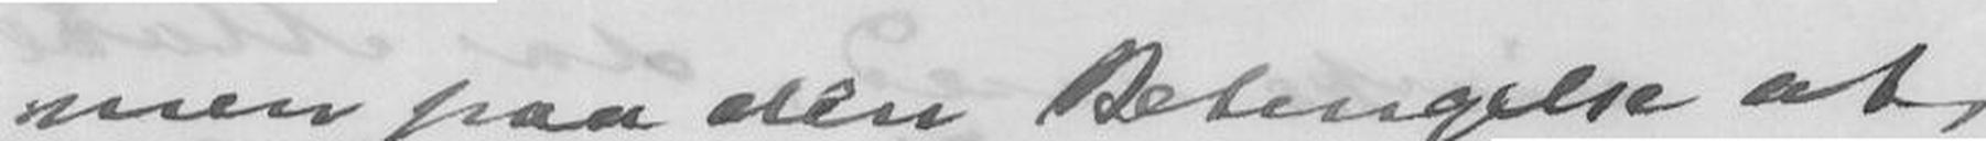men paa den Betingelse at
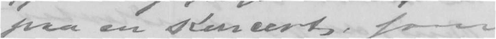paa en Koncert, som
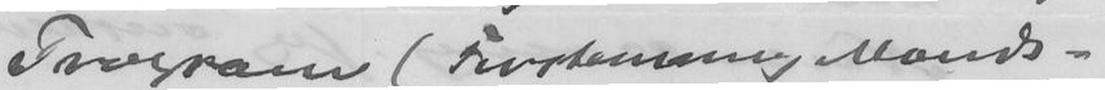Program (Firstemmig Mands-
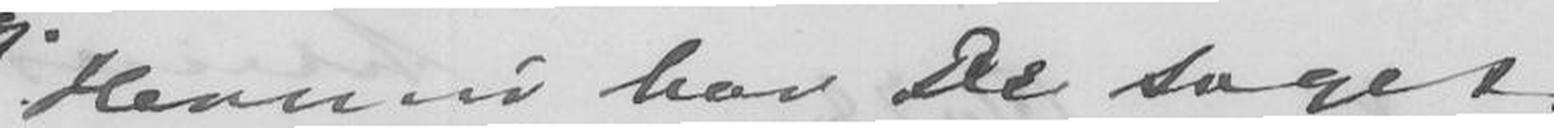Hermed har De taget
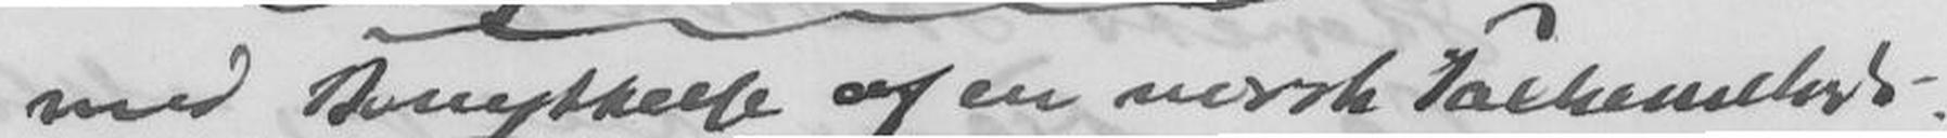med Benyttelse af en norsk Folkemelodi.
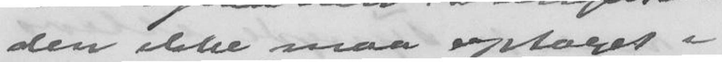den ikke maa optages i
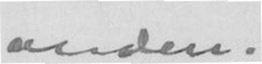anden.
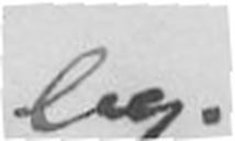lig.
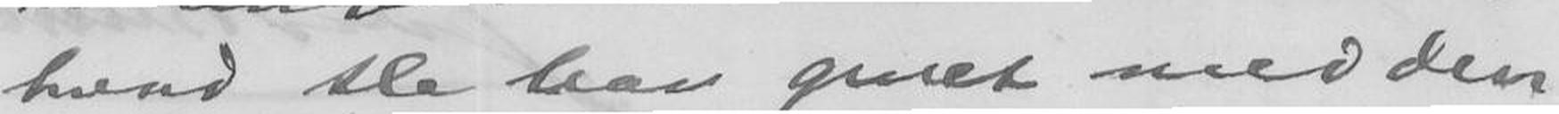hvad De har givet med den
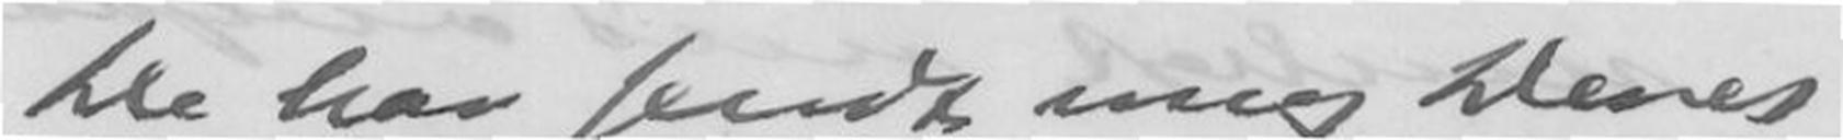De har sendt mig Deres
